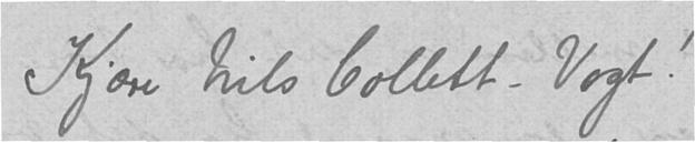Kjære Nils Collett-Vogt!
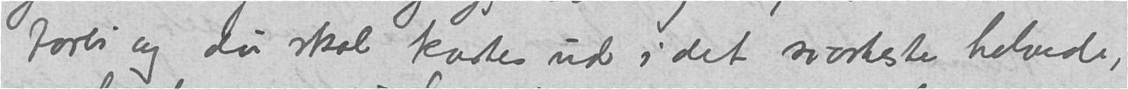forbi og du skal kastes ud i det svarteste helvede,
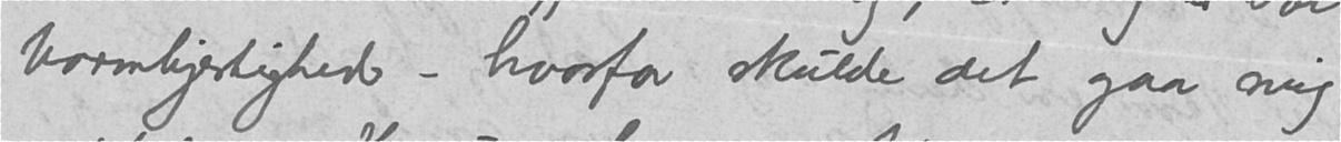barmhjertighed - hvorfor skulde det gaa mig
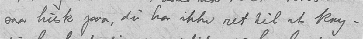saa husk paa, du har ikke ret til at kny -
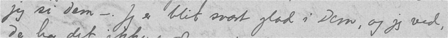jeg si dem -. Jeg er blit svært glad i Dem, og jeg ved,
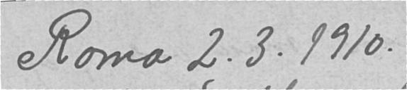Roma 2.3.1910.
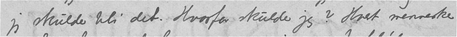jeg skulde bli det. Hvorfor skulde jeg? Hvert menneske
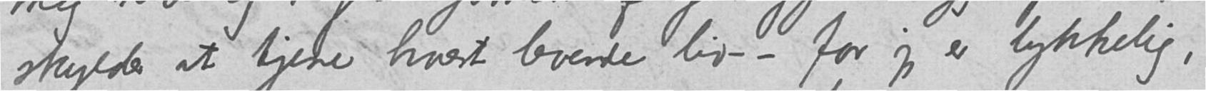skylder at tjene hvert levende liv - for jeg er lykkelig,
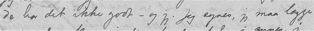De har det ikke godt - og jeg. Jeg synes, jeg maa lægge
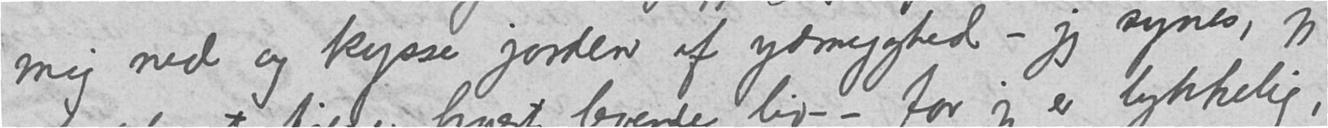mig ned og kysse jorden af ydmyghed - jeg synes, jeg
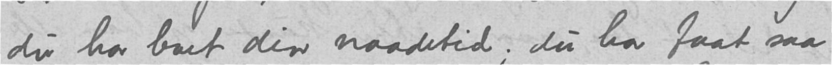du har levet din naadetid; du har faat saa
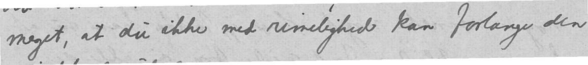meget, at du ikke med rimelighed kan forlange den
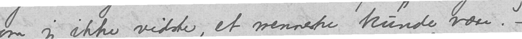som jeg ikke vidste, et menneske kunde være.
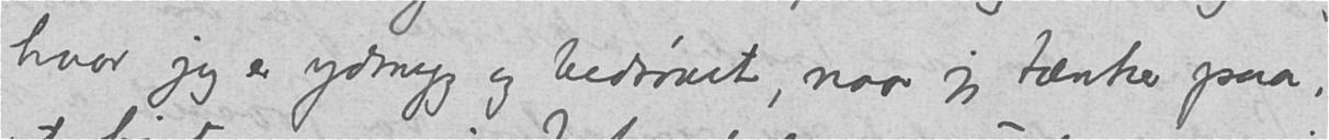hvor jeg er ydmyg og bedrøvet, naar jeg tænker paa,
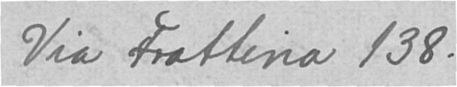Via Frattina 138.
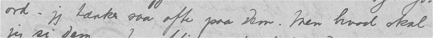ord - jeg tænker saa ofte paa dem. Men hvad skal
